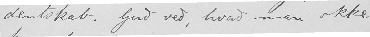dentskab. Gud ved, hvad man ikke
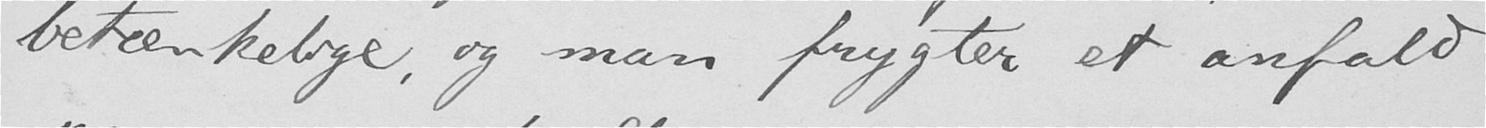betænkelige, og man frygter et anfald
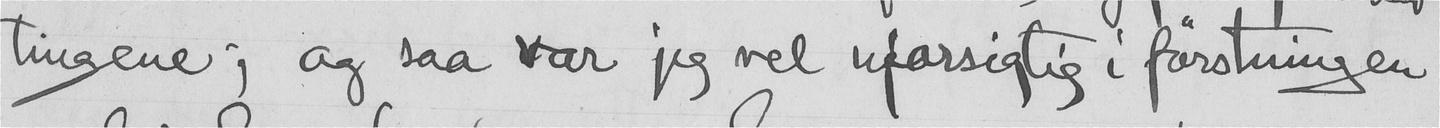tingene, og saa var jeg vel uforsigtig i førstningen
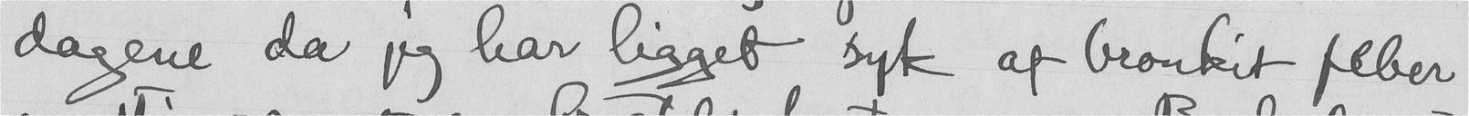dagene da jeg har ligget syk af bronkit feber
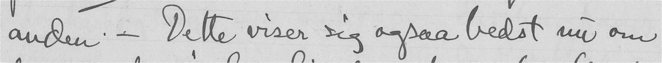anden. - Dette viser sig ogsaa bedst nu om
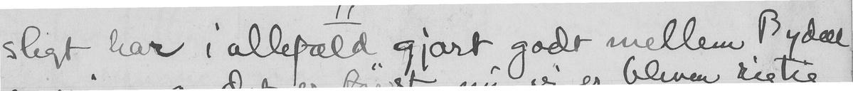sligt har i allefald gjort godt mellem Bydal
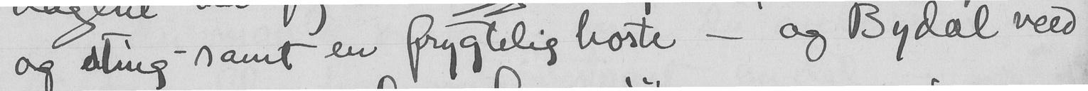og sting - samt en frygtelig hoste - og Bydal veed
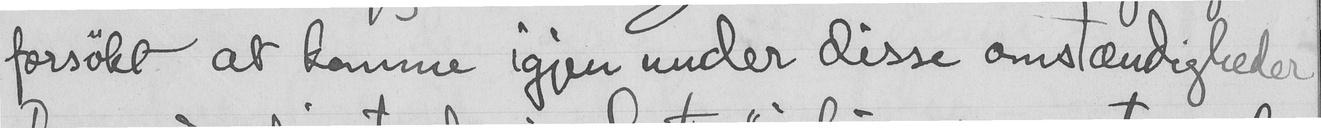forsøkt at komme igjen under disse omstændigheder
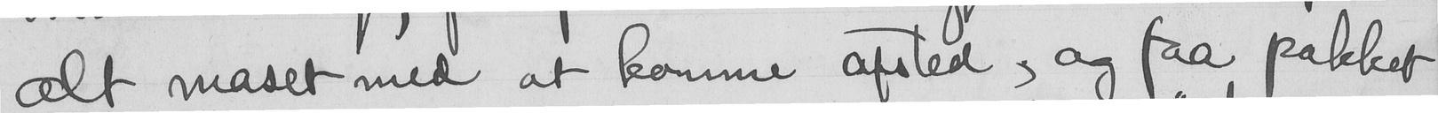alt maset med at komme afsted, og faa pakket
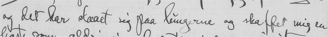og det har slaaet sig paa lungerne og skaffet mig en
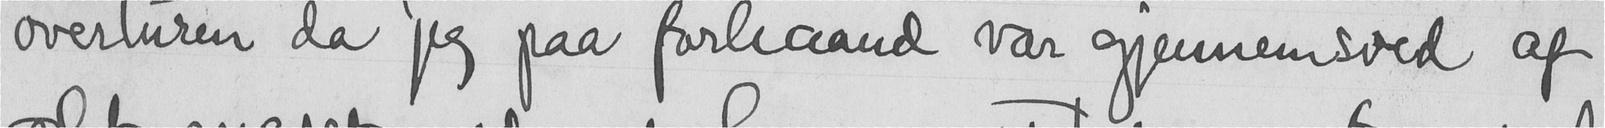overturen da jeg paa forhaand var gjennemsved af
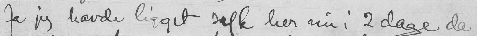Ja jeg havde ligget syk her nu i 2 dage de
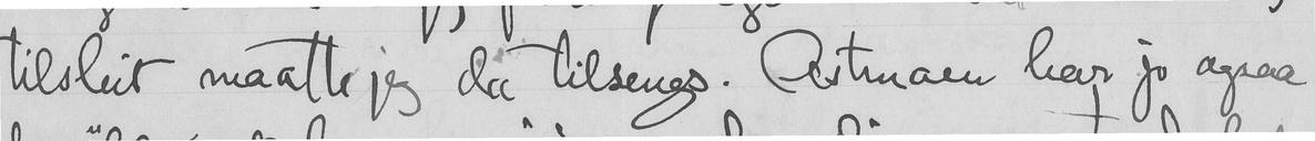tilslut maatte jeg da tilseng. Astmaen har jo ogsaa
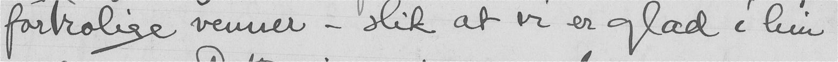fortrolige venner - slik at vi er glad i hin-
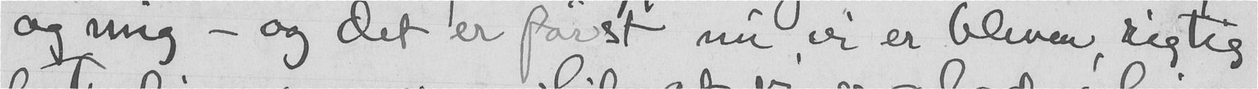og mig - og det er først nu vi er bleven rigtig
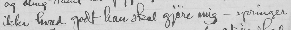ikke hvad godt han skal gjøre mig - springer
